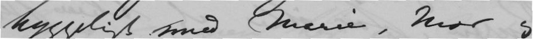hyggeligt med Marie, Mor og
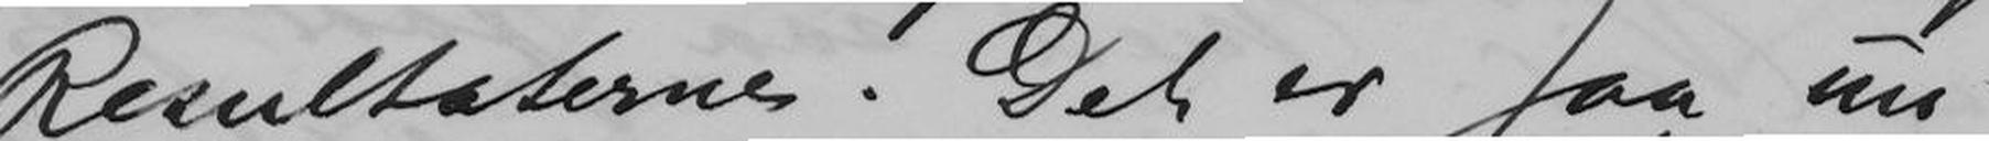Resultaterne. Det er saa un-
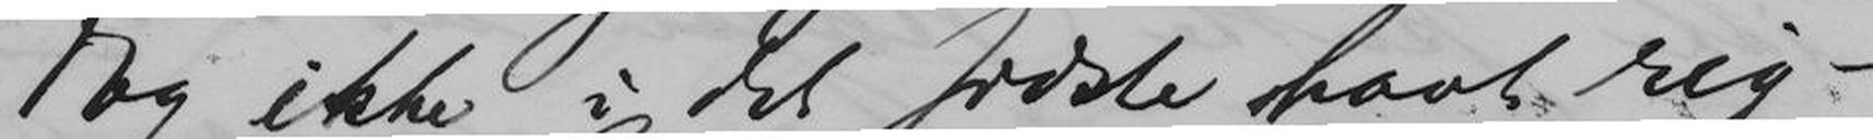dog ikke i det sidste havt rig-
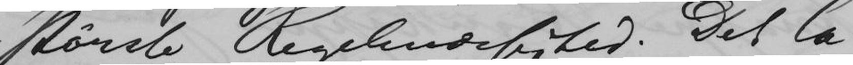største Regelmæssighed. Det la-
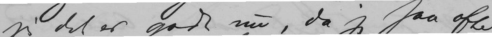jeg det er godt nu, da jeg saa ofte
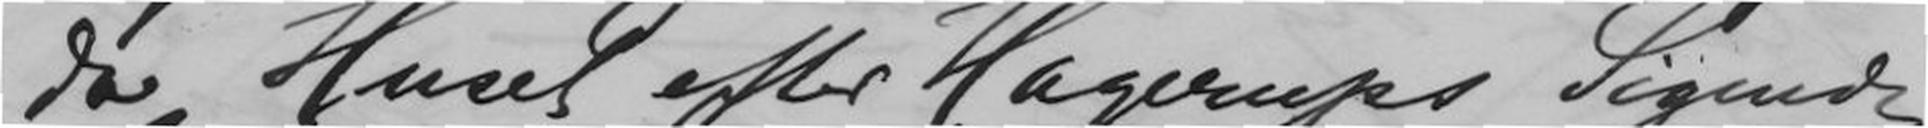da Huset efter Hagerups Sigurd
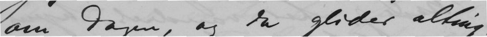om Dagen, og da glider alting.
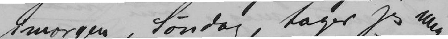imorgen, Søndag, tager jeg mig
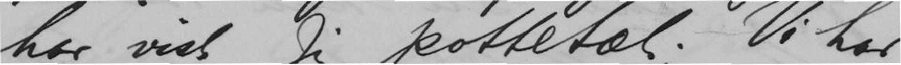har vist sig pottetæt. Vi har
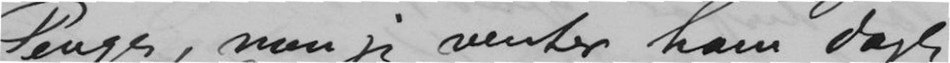Penge, men jeg venter ham daglig,
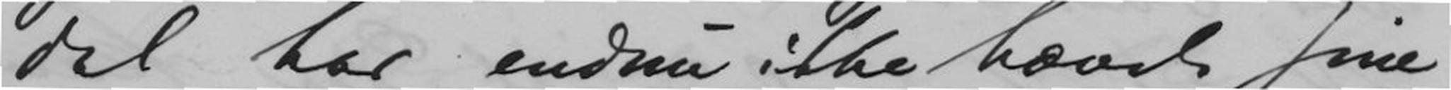dal har endnu ikke hævet sine
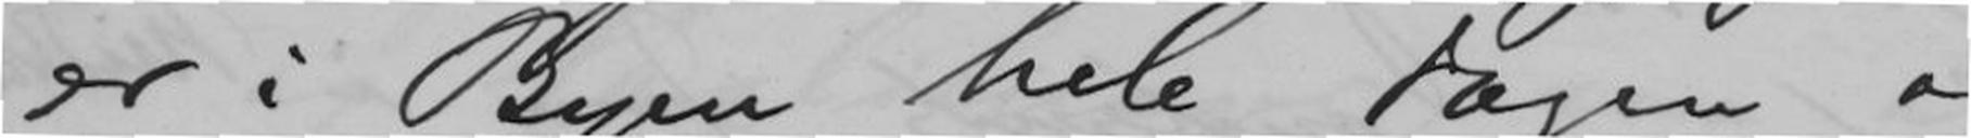er i Byen hele Dagen og
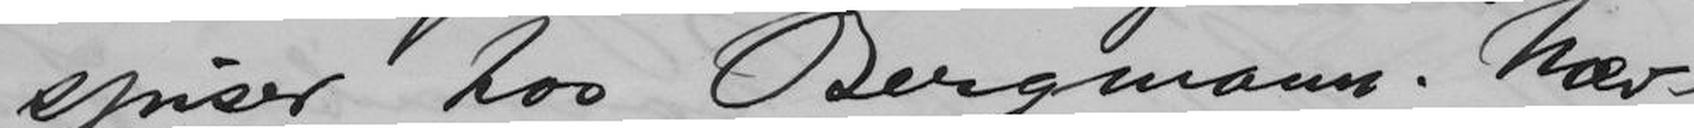spiser hos Bergmann. Næv-
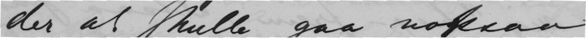der at skulle gaa noksaa
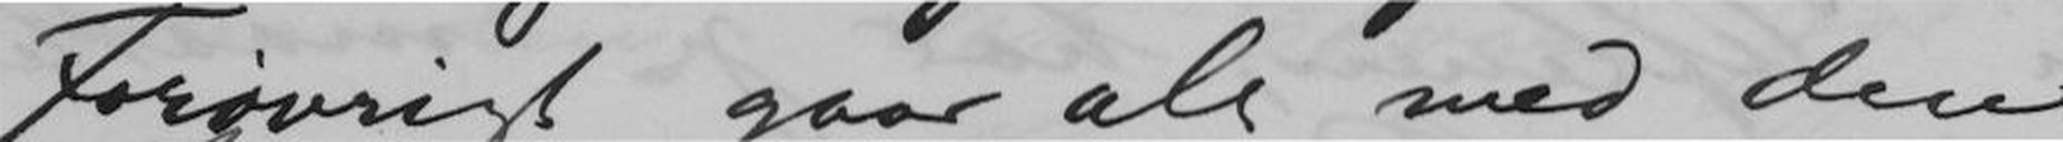Forøvrigt gaar alt med den
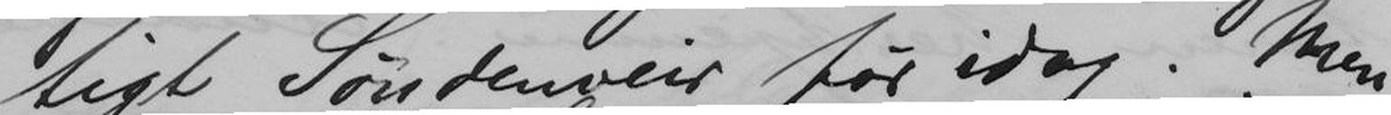tigt Søndenveir før idag. Men
